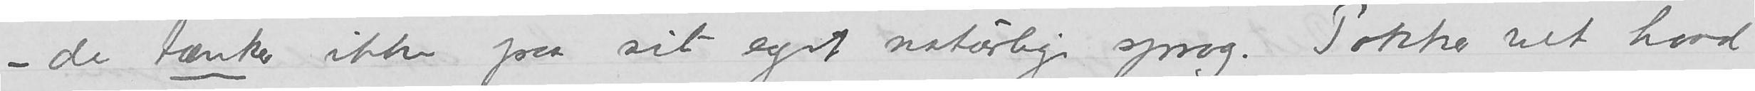– de tænker ikke paa sit eget naturlige sprog. Pokker vet hvad
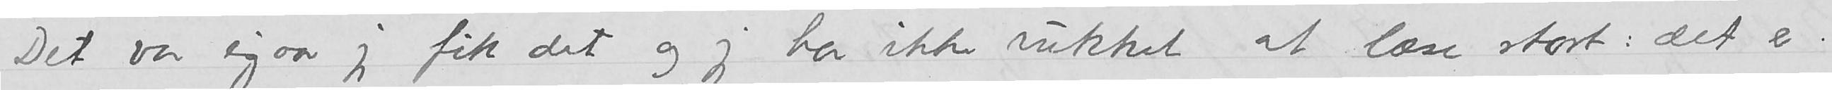Det var igaar jeg fik det og jeg har ikke rukket at læse stort: det er
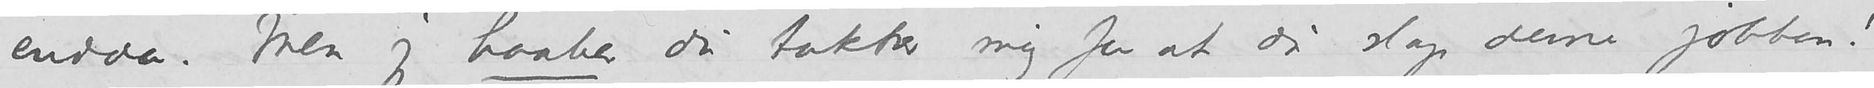endda. Men jeg haaber du takker mig for at du slap denne jobben!
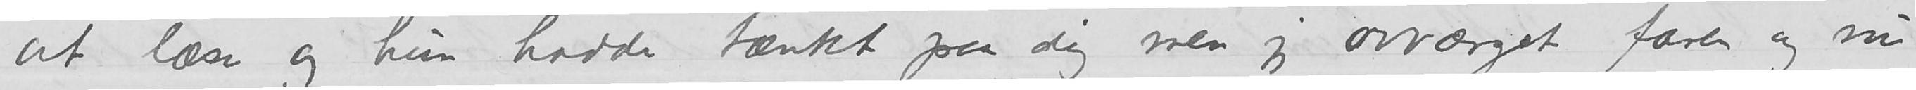at læse og hun hadde tænkt paa dig men jeg avværget faren og nu
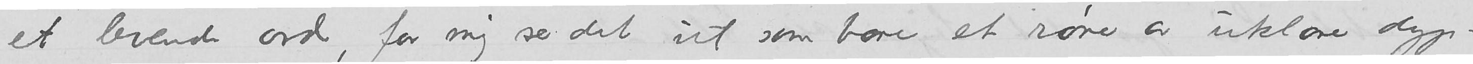et levende ord, for mig ser det ut som bare et røre av uklare dyp-
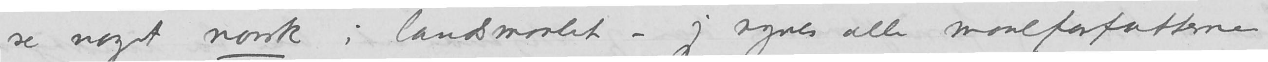se noget norsk i landsmaalet – jeg synes alle maalforfatterne
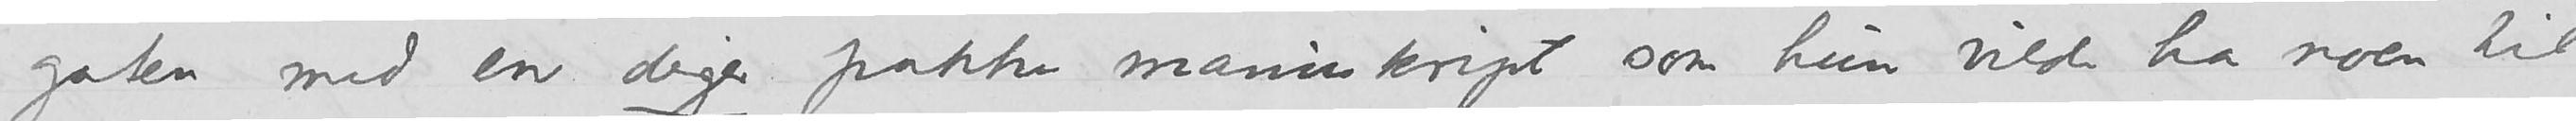gaten med en diger pakke manuskript som hun vilde ha noen til
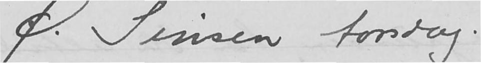Ø. Sinsen torsdag.
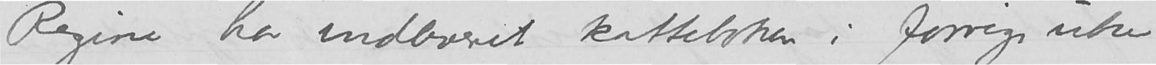Regine har indleveret katteboken i forrige uke
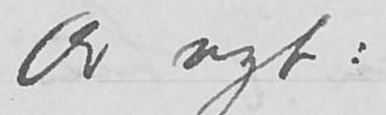Av nyt:
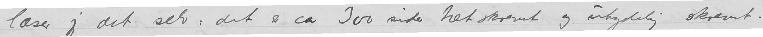læser jeg det selv: det er ca 300 sider tætskrevet og utydelig skrevet.
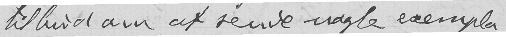tilbud om at sende nogle exempla-
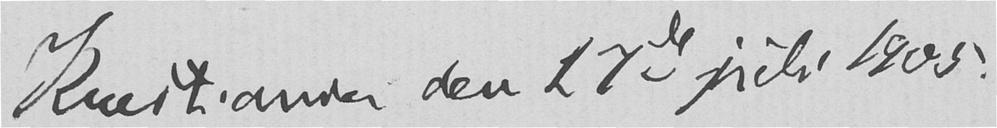Kristiania den 17de juli 1905.
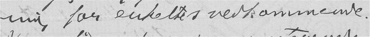mig for enkeltes vedkommende.
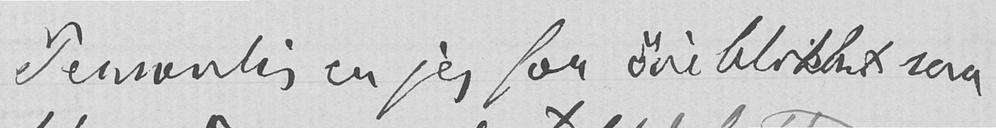Personlig er jeg for øieblikket saa
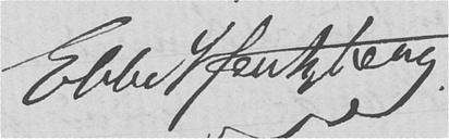Ebbe Hertzberg.
